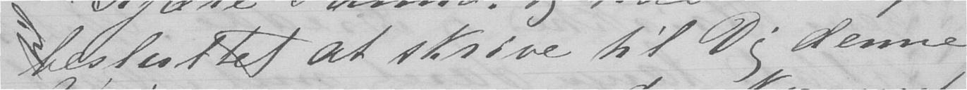besluttet at skrive til Dig denne
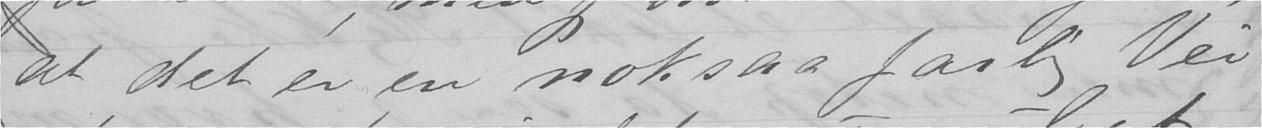at det er en noksaa farlig Vei
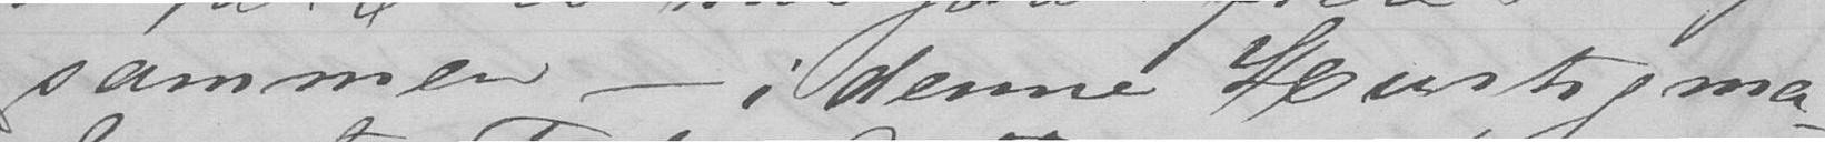sammen - i denne Hurtigma-
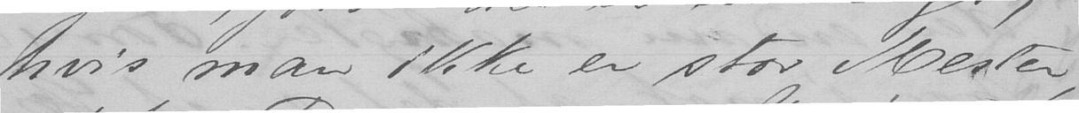hvis man ikke er stor Mester,
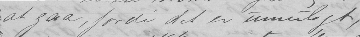at gaa, fordi det er umuligt,
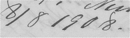8/8 1908.
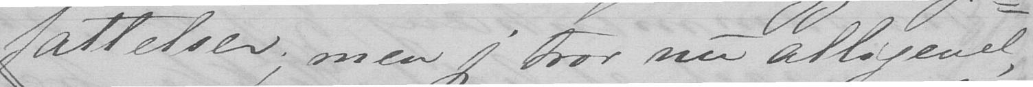fattelser; men jeg tror nu alligevel
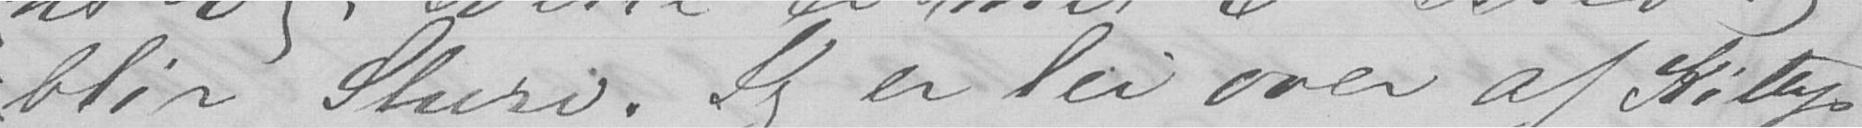blir Slurv. Jeg er lei over af Kittys
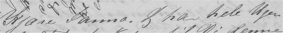Kjære Janna. Jeg har hele Ugen
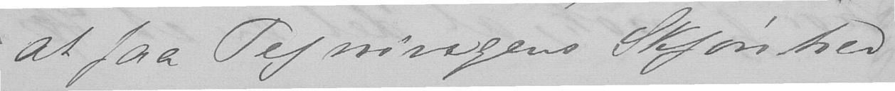at faa Tegningens Skjønhed
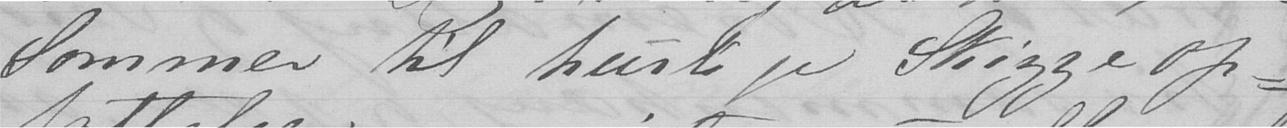Sommer til huslige Skizzeop-
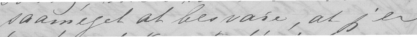saameget at besvare, at jeg er
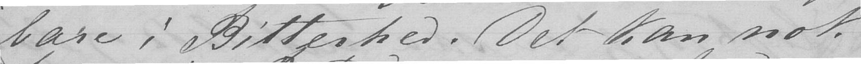bare i Bitterhed. Det kan nok
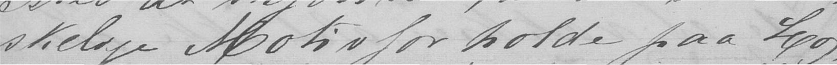skelige Motivforholde paa Hog-
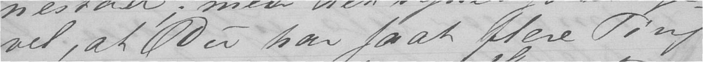vel, at Du har faat flere Ting
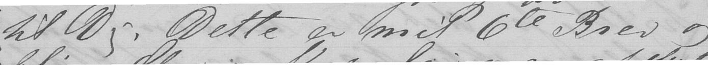til Dig. Dette er mit 6te Brev og
